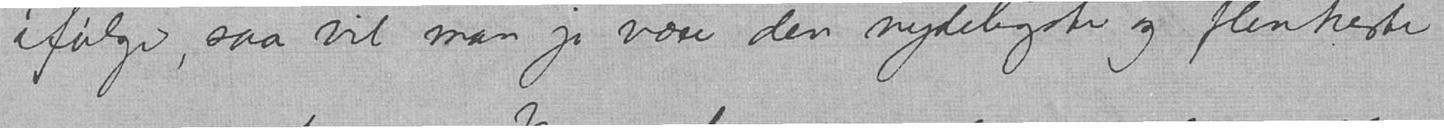ifølge, saa vil man jo være den nydeligste og flinkeste
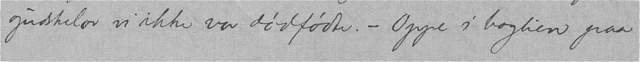gudskelov vi ikke var dødfødte. - Oppe i baglien paa
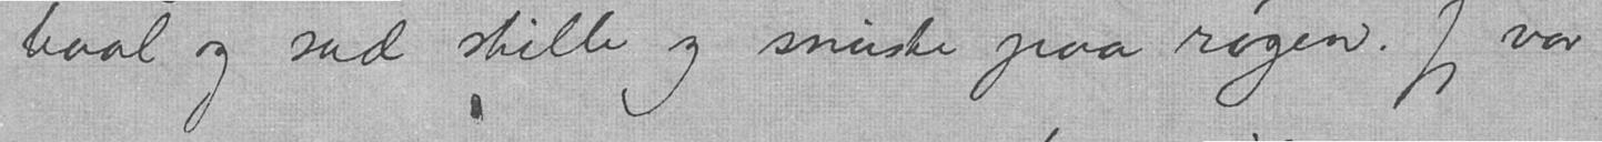baal og sad stille og snuste paa røgen. Jeg var
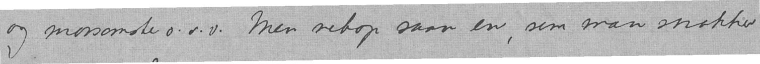og morsomste o.s.v. Men netop saan en, som man snakker
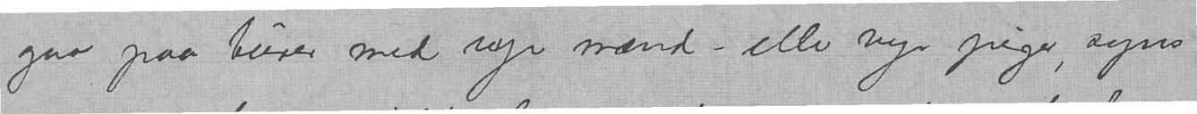gaa paa turer med nye mænd - eller nye piger, syns
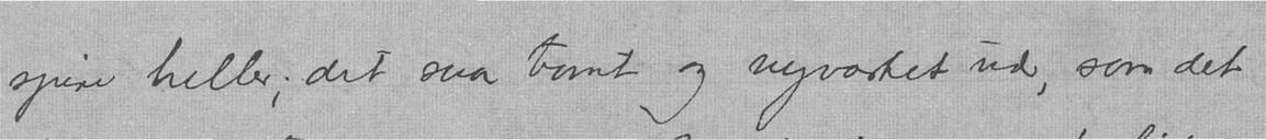spire heller; det saa tomt og nyvasket ud, som det
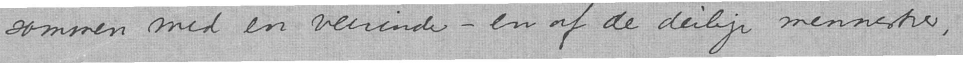sammen med en veninde - en af de deilige mennesker,
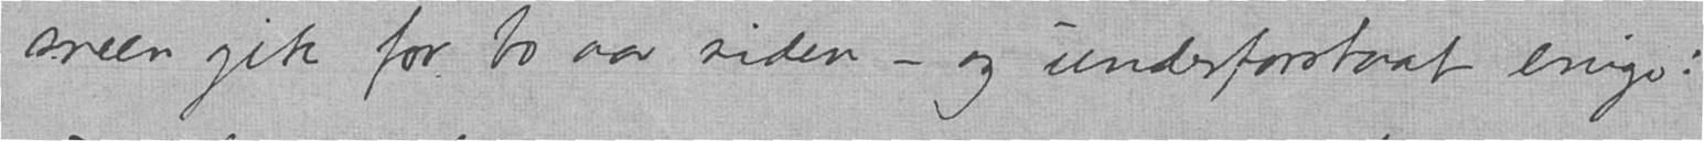sneen gik for to aar siden - og underforstaat enige!
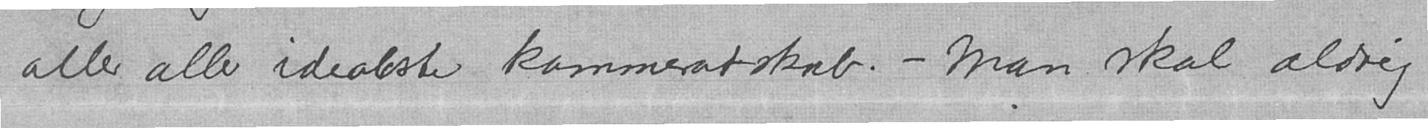aller aller idealeste kammeratskab. - Man skal aldrig
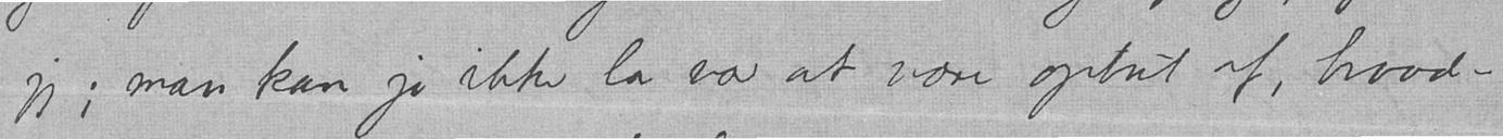jeg; man kan jo ikke la være at være optat af, hvad-
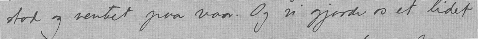stod og ventet paa vaar. Og vi gjorde os et lidet
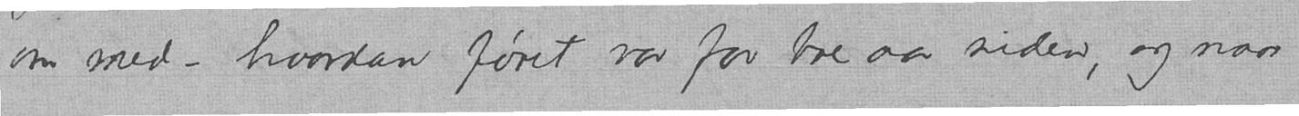om med - hvordan føret var for tre aar siden, og naar
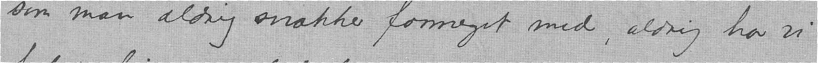som man aldrig snakker formeget med, aldrig har vi
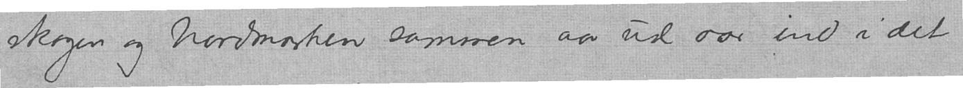skogen og Nordmarken sammen aar ud aar ind i det
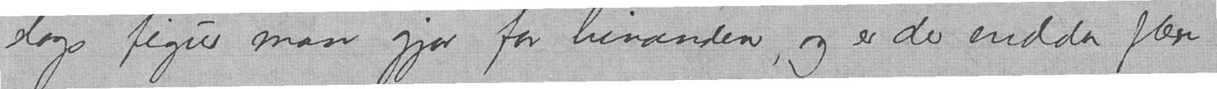slags figur man gjør for hinanden, og er de endda flere
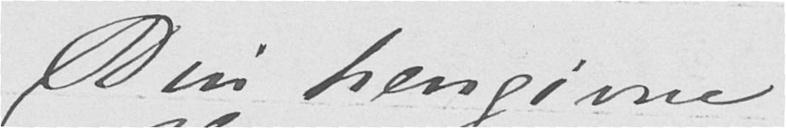Din hengivne
